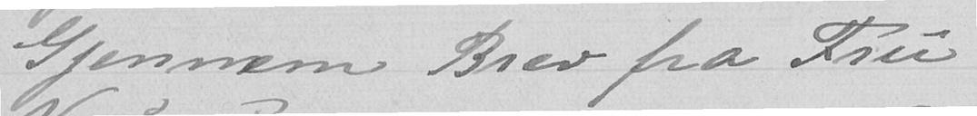Gjennem Brev fra Fru
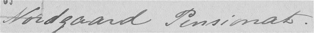Nordgaard Pensionat.
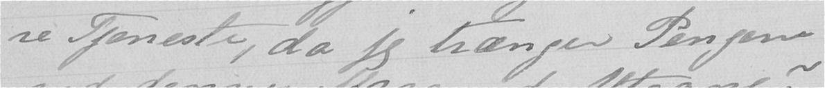re Tjeneste, da jeg trænger Pengene
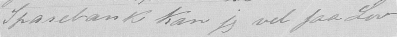Sparebank kan jeg vel faa Lov,
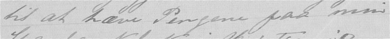til at hæve Pengene paa min
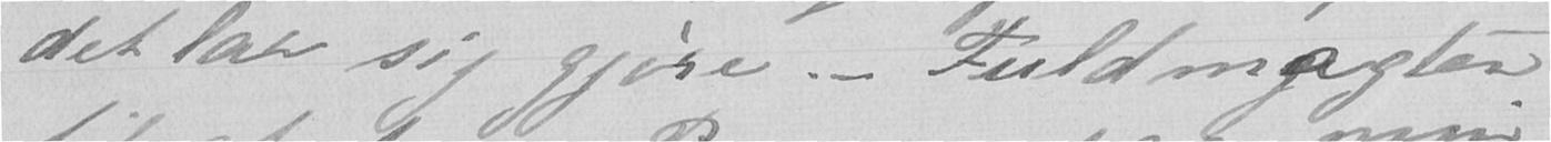det lar sig gjøre. - Fuldmagten
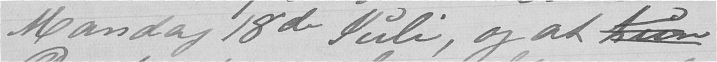Mandag 18de Juli, og at ture
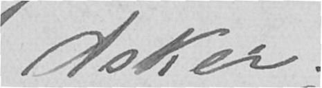Asker.
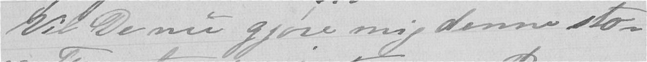Vil De nu gjøre mig denne sto-
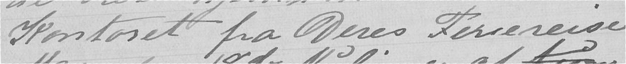Kontoret fra Deres Feriereise
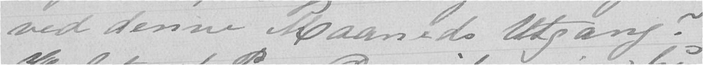ved denne Maaneds Utgang?
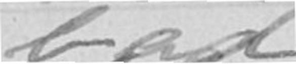bad
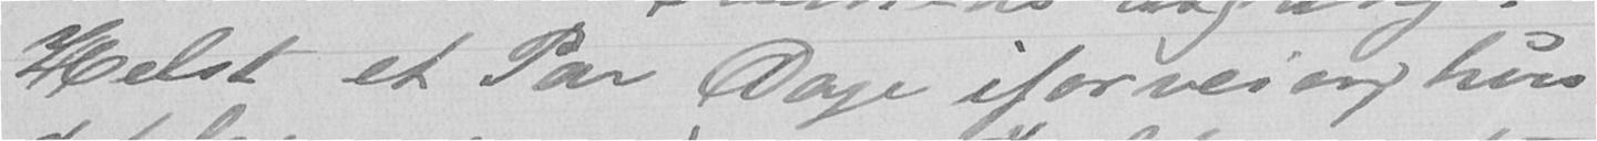Helst et Par Dage iforveien, hvis
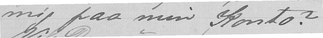mig paa min Konto?
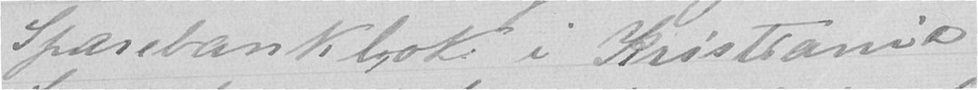Sparebankbok i Kristiania
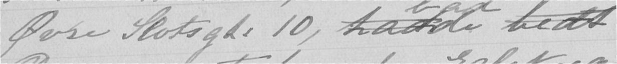Øvre Slotsgt. 10, hadde bedt
